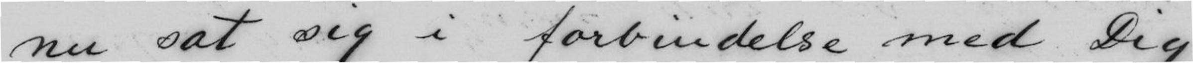nu sat sig i forbindelse med Dig
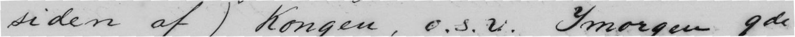siden af) Kongen, o.s.v. Imorgen 9de
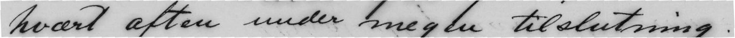hvært aften under megen tilslutning.
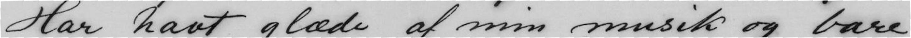Har havt glæde af min musik og bare
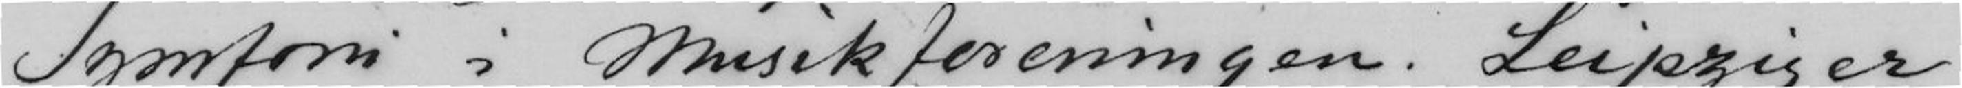Symfoni i Musikforeningen. Leipziger
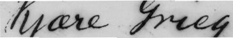Kjære Grieg
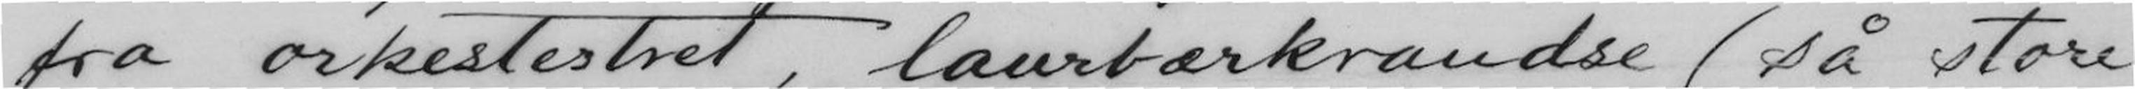fra orkestestret, laurbærkrandse (så store
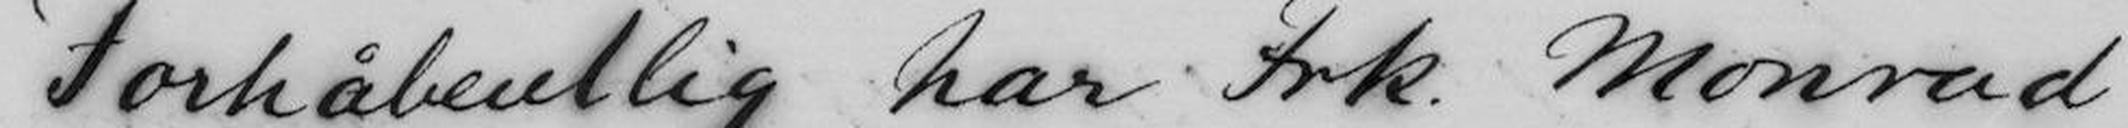Forhåbentlig har Frk. Monrad
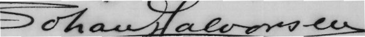Johan Halvorsen
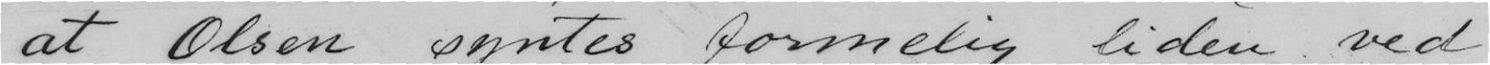at Olsen syntes formelig liden ved
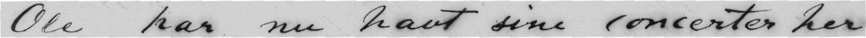Ole har nu havt sine concerter her
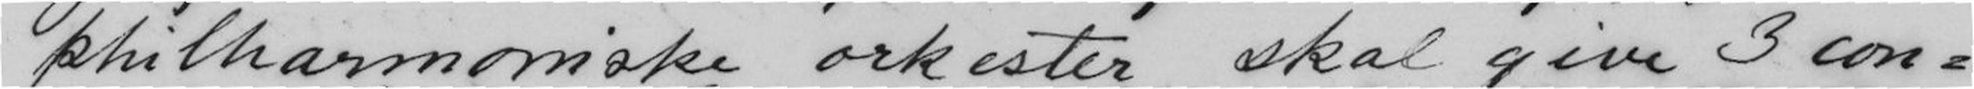philharmoniske orkester skal give 3 con-
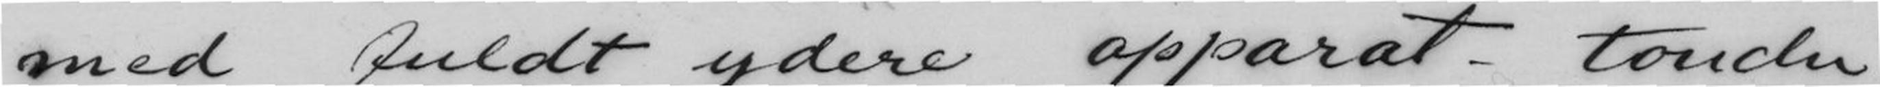med fuldt ydere apparat- touche
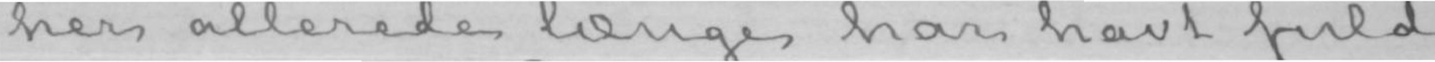her allerede længe har havt fuld
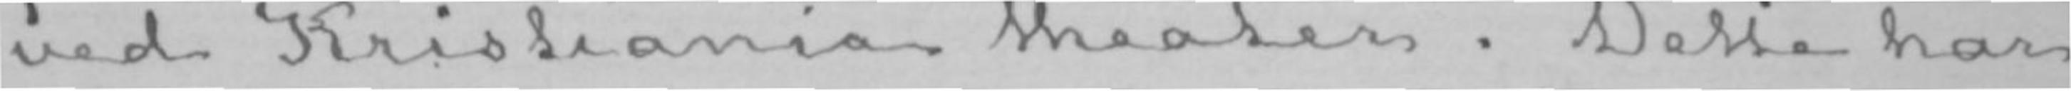ved Kristiania theater. Dette har
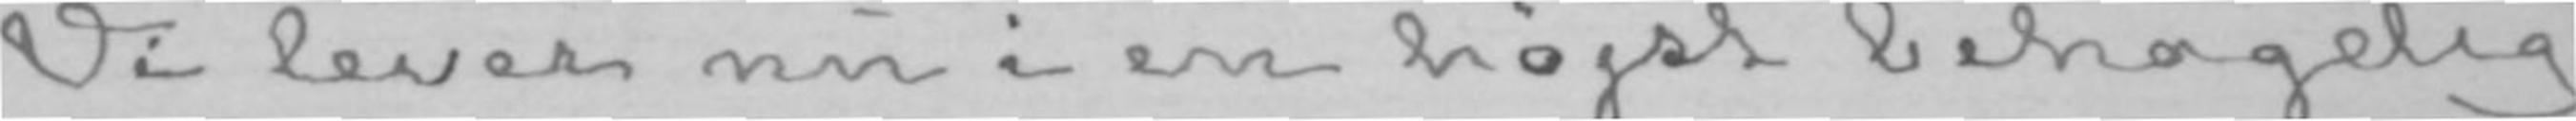Vi lever nu i en højst behagelig
